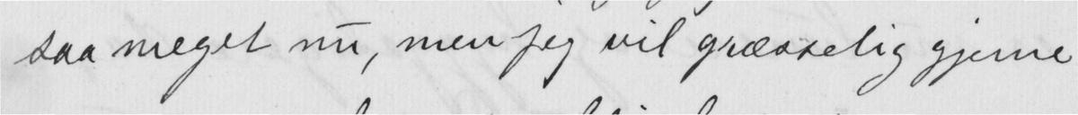saa meget nu, men jeg vil græsselig gjerne
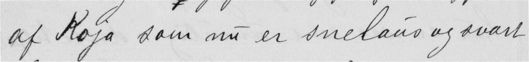af Koja som nu er snelaus og svart
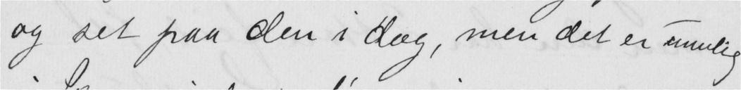og det paa den i dag, men det er umulig
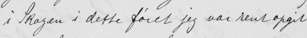i Skogen i dette føret jeg var rent opgit
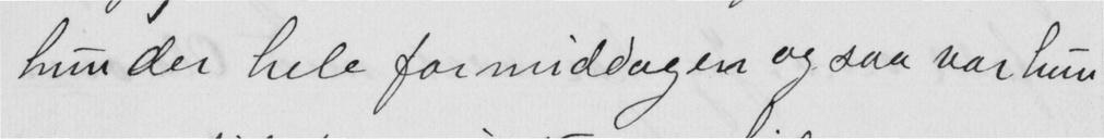hun der hele formiddagen og saa var hun
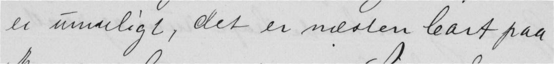er umuligt, det er næsten bart paa
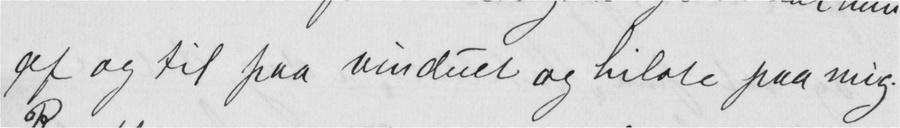af og til paa vinduet og hilste paa mig.
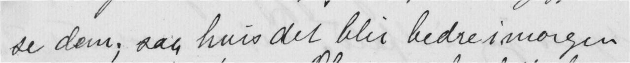se dem, sag hvis det blev bedre imorgen
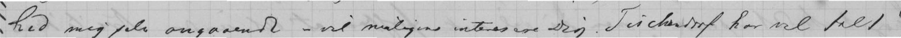hed mig selv omgaaende - vil muligens interessere Dig. Tischendorf har vel talt
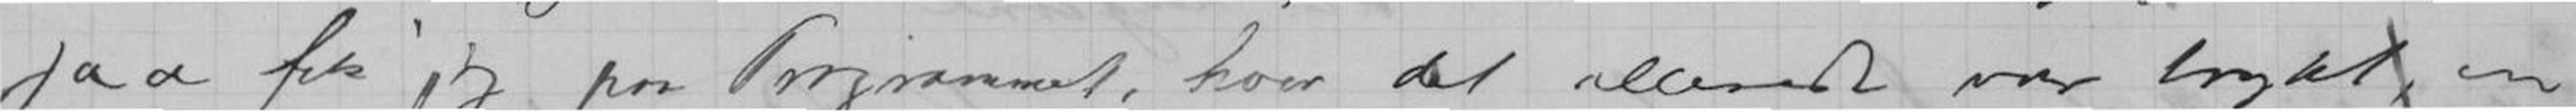saa fik jeg paa Programmet, hvor det Allerede var trykt en
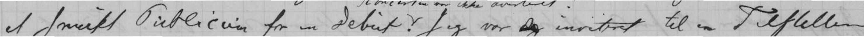et smukt Publicum for en Debut. Jeg var inviteret til en Tilstelling
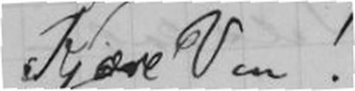Kjære Ven!
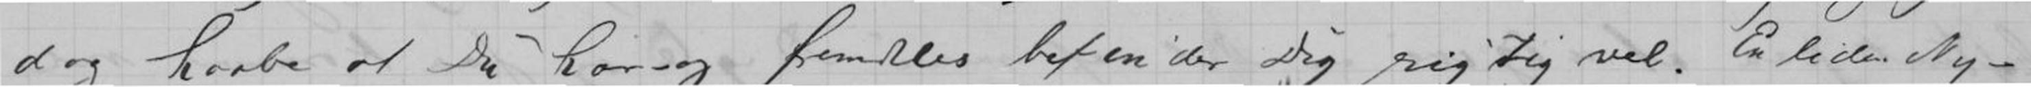dog haabe at Du har og fremdeles befinder dig rigtig vel. En liden Ny-
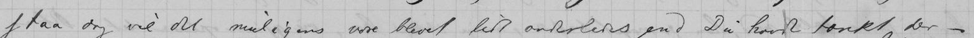staa dog vil det muligens være blevet lidt anderledes end Du havde tankt der-
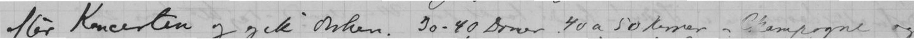efter Koncerten og gik dithen. 30-40 Damer. 40 a 50 Herrer Champagne og
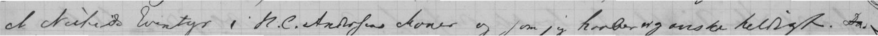et Nutids Eventyr i H. C. Andersens koner og som jeg haaber er ganske heldigt. Da
